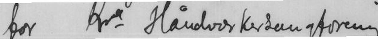for Kra Håndværkersangforening
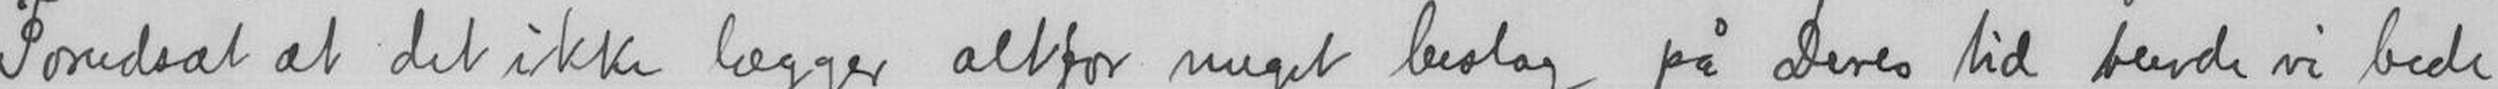Forudsat at det ikke lægger altfor meget beslag på Deres tid turde vi bede
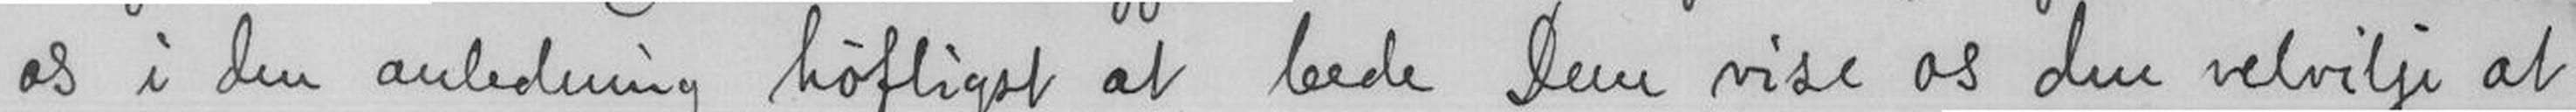os i den anledning høfligst at bede Dem vise os den velvilje at
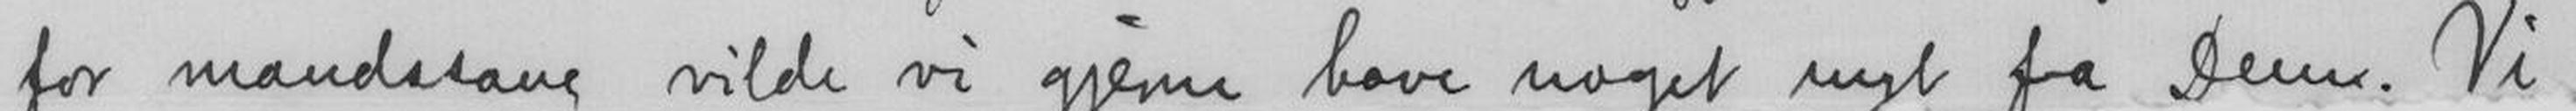for mandssang vilde vi gjerne have noget nyt fra Dem. Vi
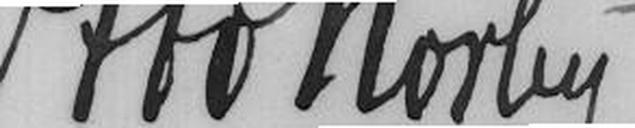Otto Norby
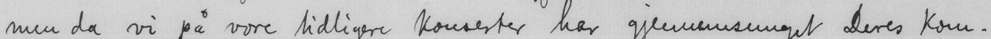men da vi på vore tidligere konserter har gjennemsunget Deres kom
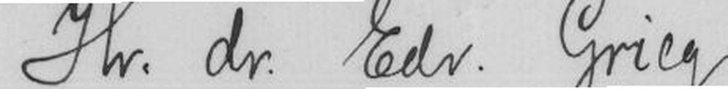Hr. dr. Edv. Grieg
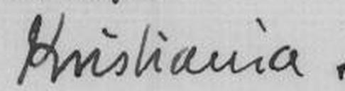Kristiania.
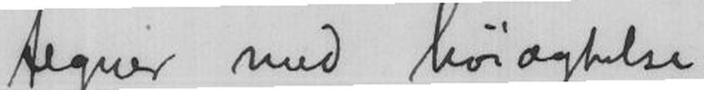tegner med høiagtelse
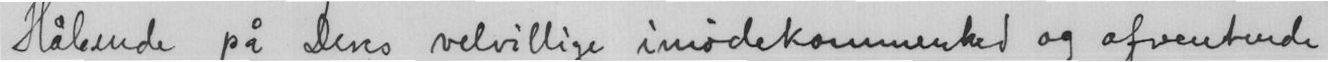Håbende på Deres velvillige imødekommenhed og afventende
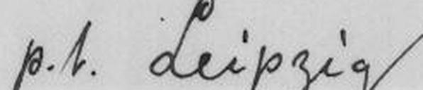p.t. Leipzig
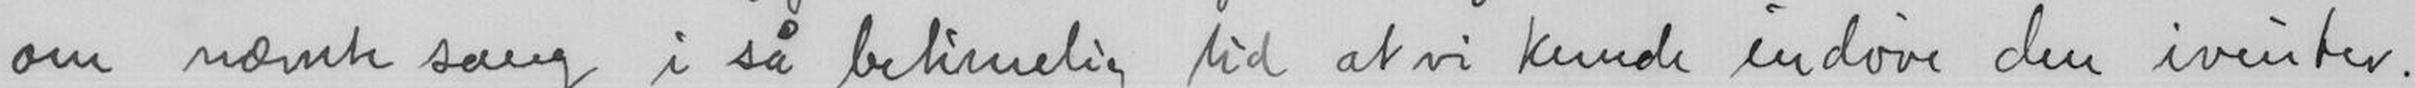om nævnte sang i så betimelig tid at vi kunde indøve den ivinter.
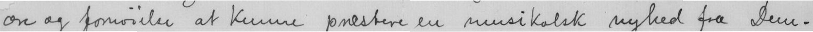ære og fornøielse at kunne præstere en musikalsk nyhet fra Dem.
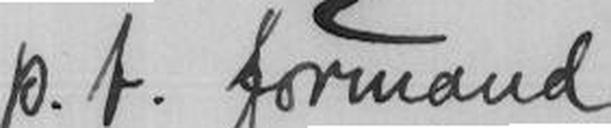p.t. formand
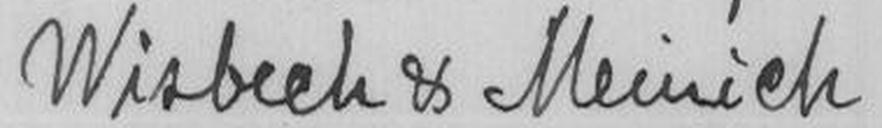Wisbech & Meinich
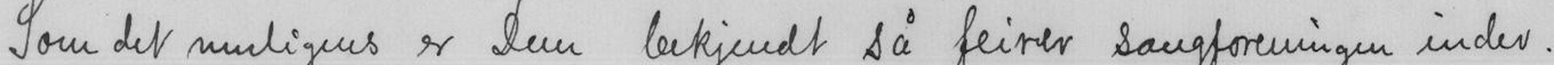Som det muligens er Dem bekjendt så feirer sangforeningen inden
